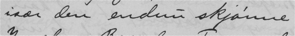især den endnu skjønne
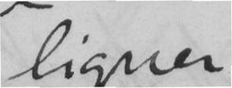ligner
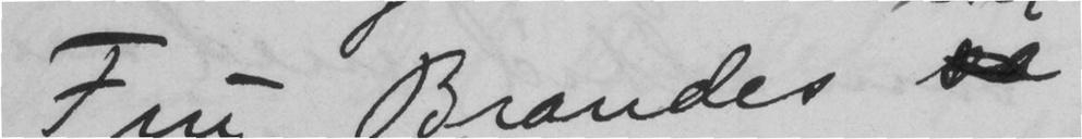Fru Brandes
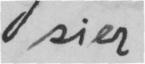sier
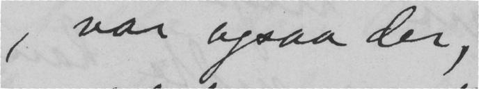, var ogsaa der,
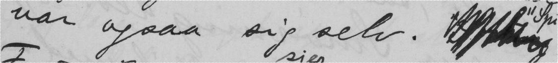var ogsaa sig selv.
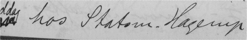hos Statsm. Hagerup
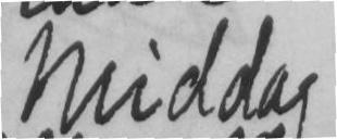middag
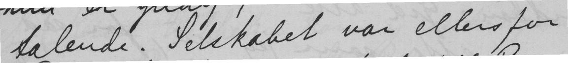talende. Selskabet var ellers for
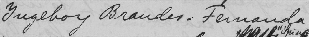Ingeborg Brandes. Fernanda
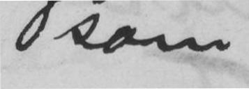som
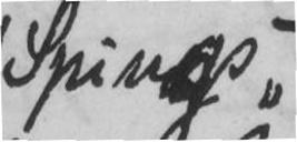"Spings"
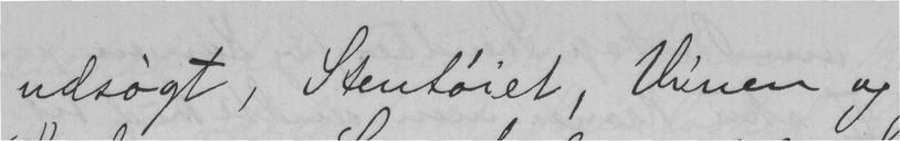udsøgt, Stentøiet, Vinen og
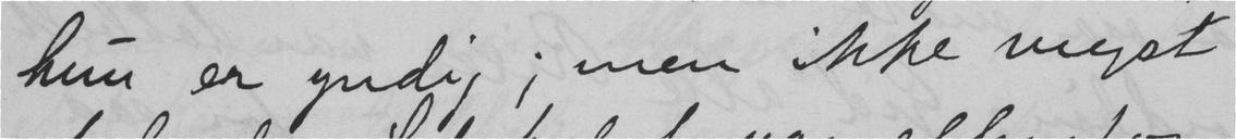hun er yndig; men ikke meget
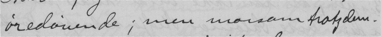øredøvende; men morsom trotz dem.
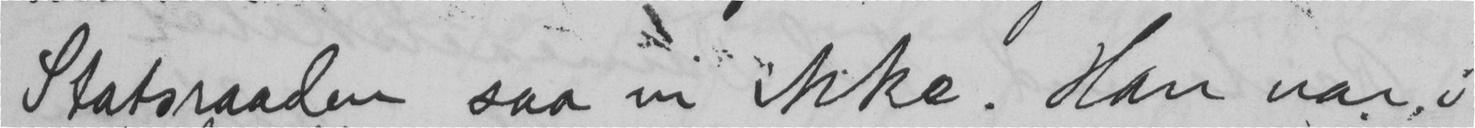Statsraaden saa vi ikke. Han var i
